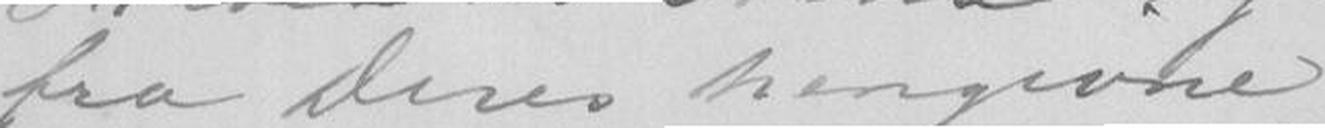fra Deres hengivne
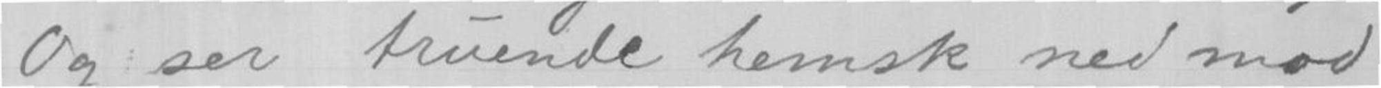Og ser truende hemsk ned mod
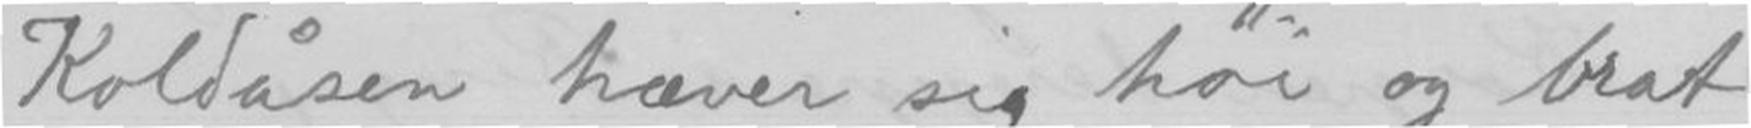Koldåsen hæver sig høi og brat
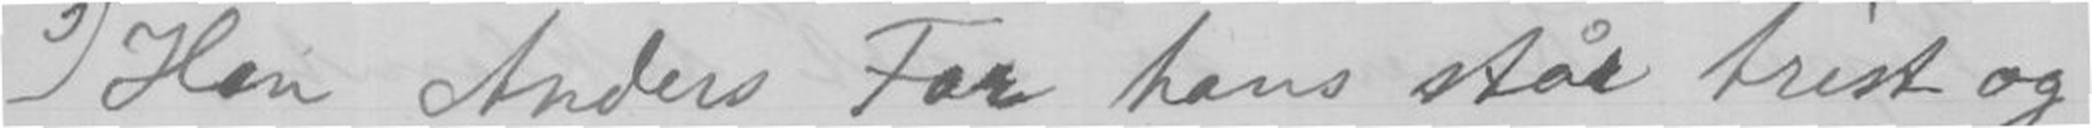Han Anders Far hans står trist og stum
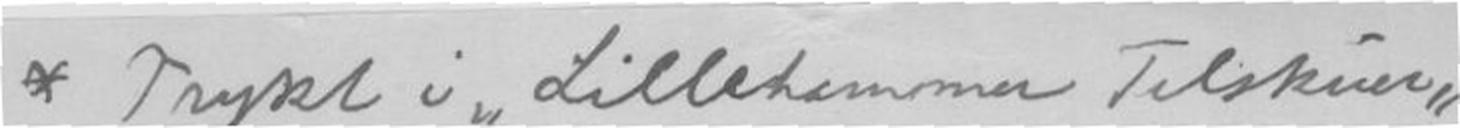* Trygt i "Lillehammer Tilskuer"
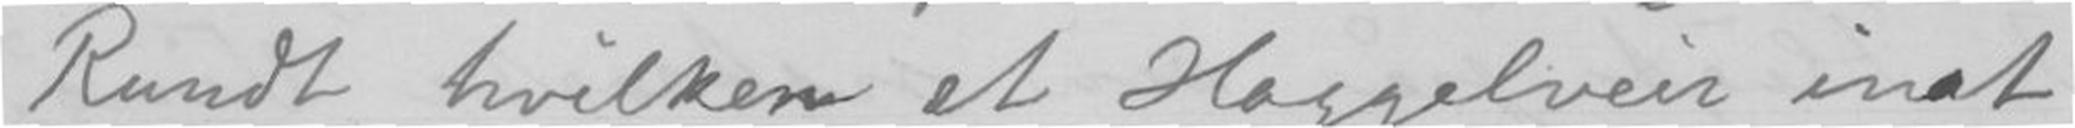Rundt hvilken et Haggelveir inst
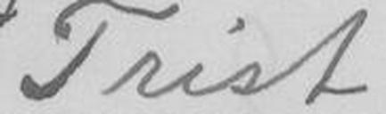* Trist
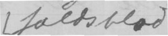faldsbad
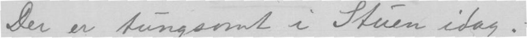Der er tungsomt i Stuen idag.
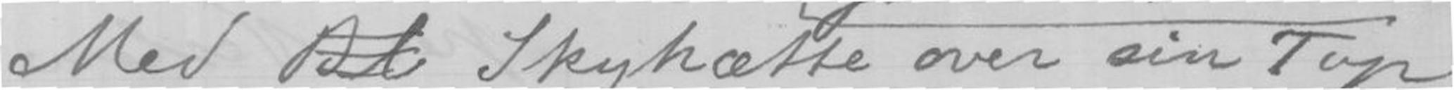Med Skyhætte over sin Top
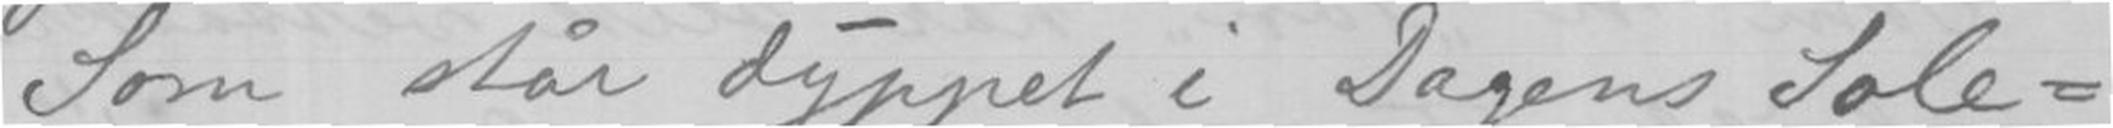Som står dyppet i Dagens Sole-
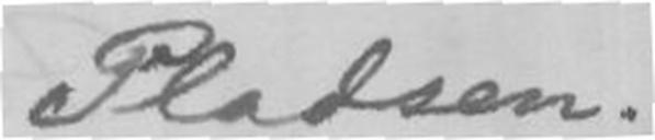Pladsen.
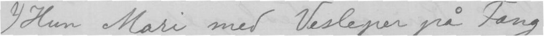Hun Mari med Vesleper på Fang
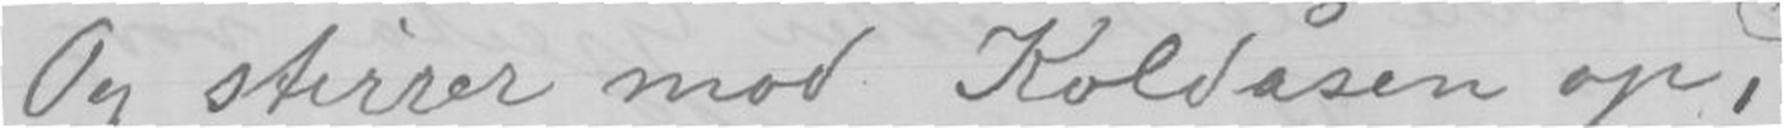Og stirrer mod Koldåsen op,
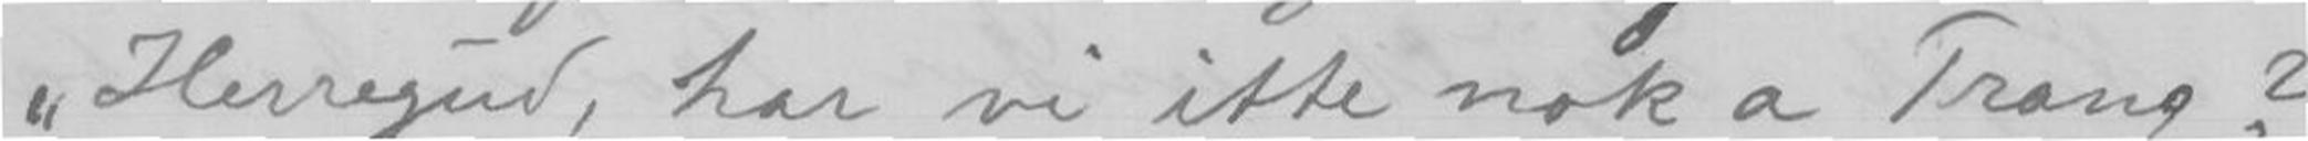"Herregud, har vi itte nok a Trang?
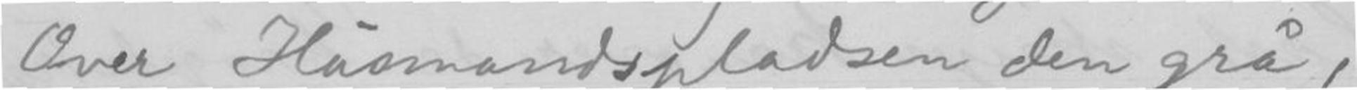Over Husmandspladsen den grå,
